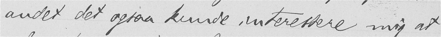andet det ogsaa kunde interessere mig at
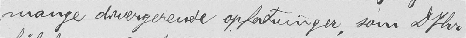mange divergerende opfatninger, som D Ihr
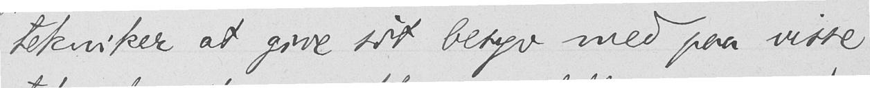tekniker at give sit besyv med paa visse
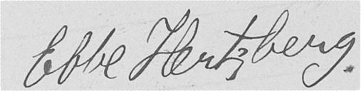Ebbe Hertzberg.
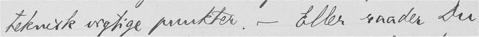teknisk vigtige punkter. - Eller raader Du
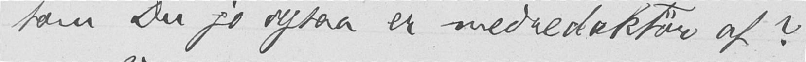som Du jo ogsaa er medredaktør af?
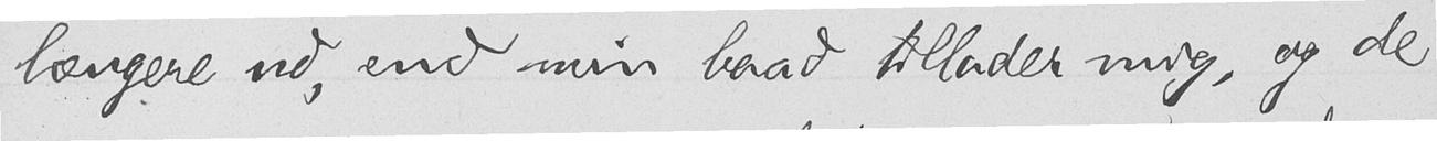længere ud, end min baad tillader mig, og de
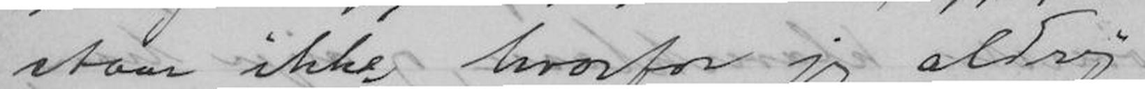taar ikke hvorfor jeg aldrig
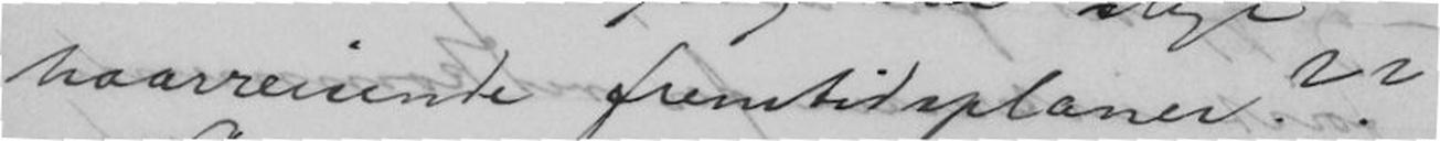haarreisende fremtidsplaner??
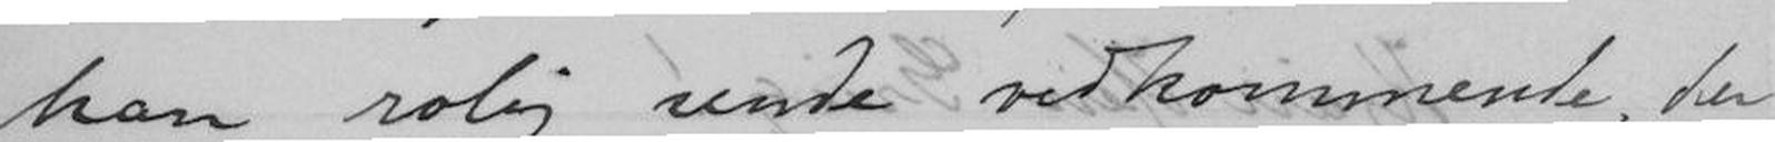kan rolig sende vedkommende, der
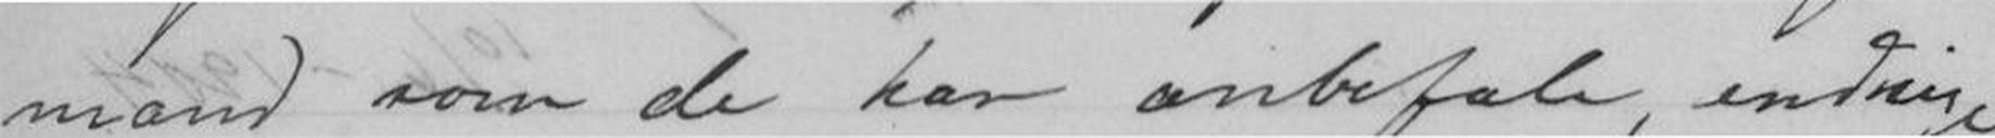mand som de kan anbefale, endrige
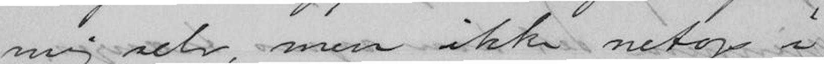mig selv, men ikke netop i
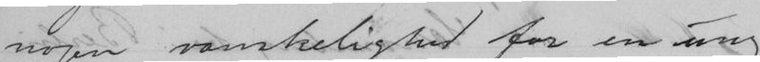nogen vanskelighed for en ung
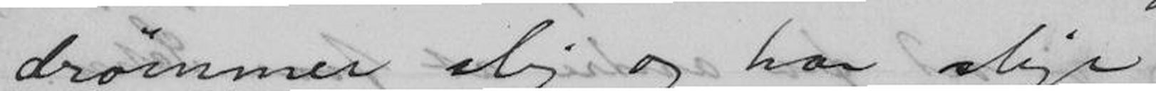drømmer slig og har slige
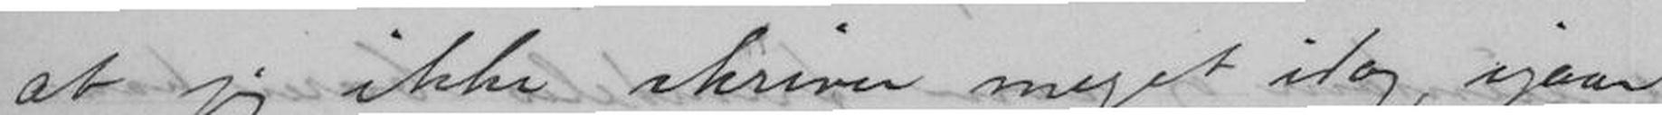at jeg ikke skriver meget idag, igaar
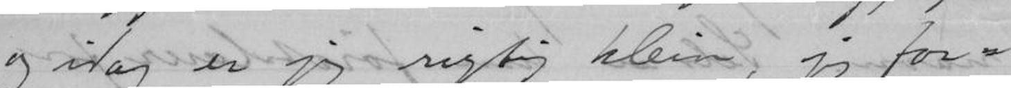og idag er jeg rigtig klein, jeg for-
